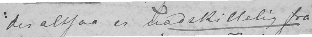der altsaa er uadskillelig fra
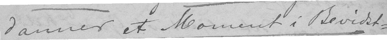danner et Moment i Bevidst-
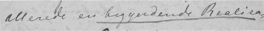allerede en begyndende Realisa-
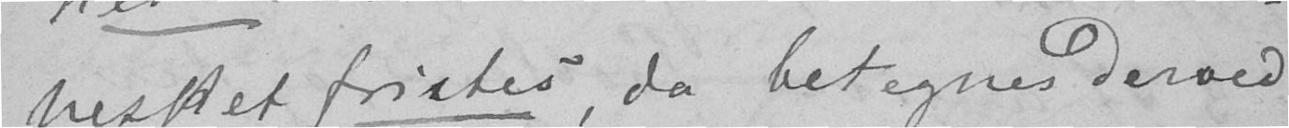nesket fristes, da betegnes derved
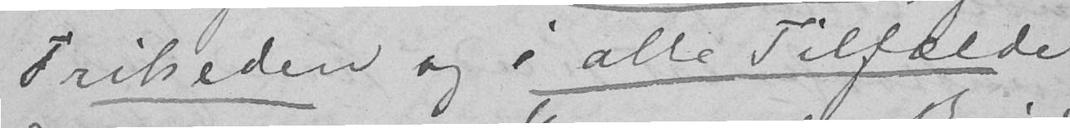Friheden og i alle Tilfælde
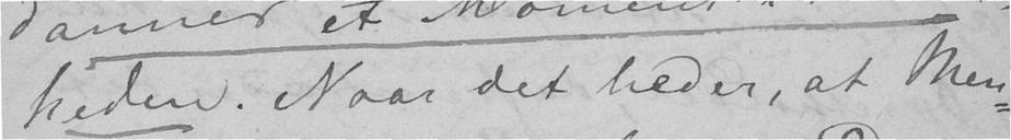heden. Naar det heder, at Men-
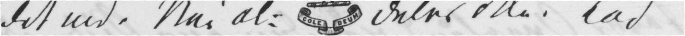det ud. Nei aldeles ikke. Lad
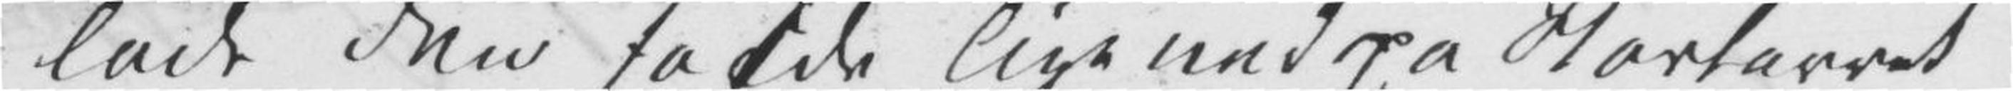lade den falde lige ned på Stortorvet
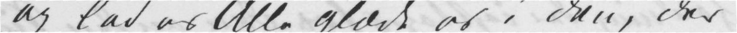og lad os Alle glæde os i den, der
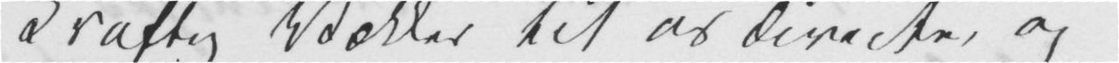kraftig Vækker til os directe, og
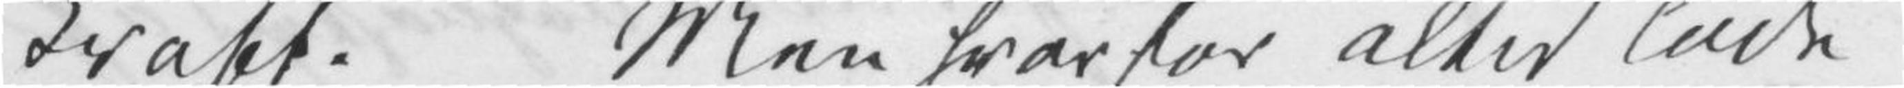Kraft. Men hvorfor altid lade
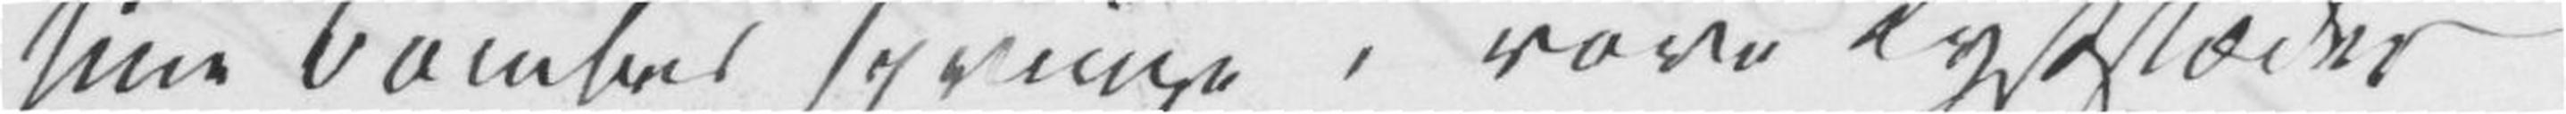sine Bomber springe i vore Kyststæder
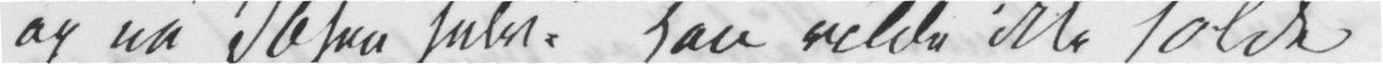og nu Ibsen selv! Han vilde ikke holde
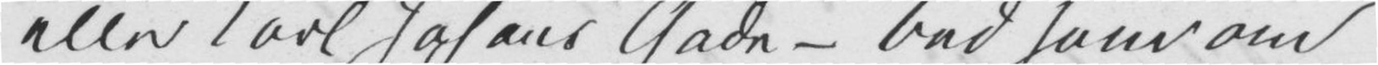eller Karl Johans Gade – bed ham om
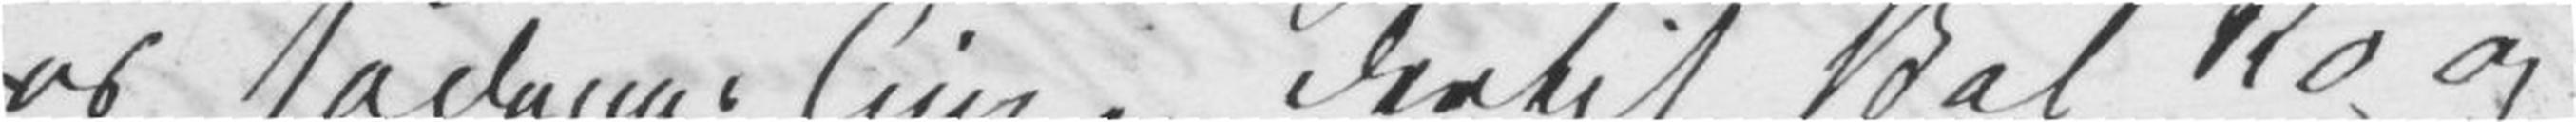os sådanne Ting. Dertil skal Ro og
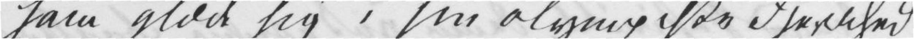ham glæde sig i sin olympiske Fjernhed
